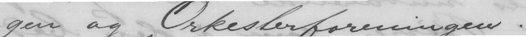gen og Orkesterforeningen.
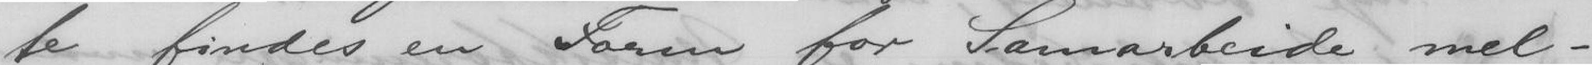te findes en Form for Samarbeide mel-
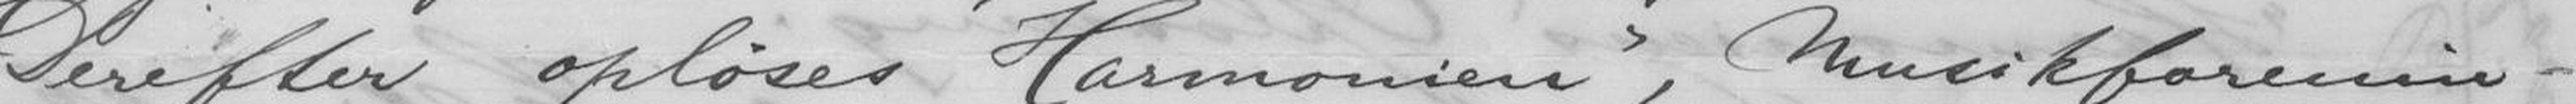Derefter opløses "Harmonien", Musikforenin-
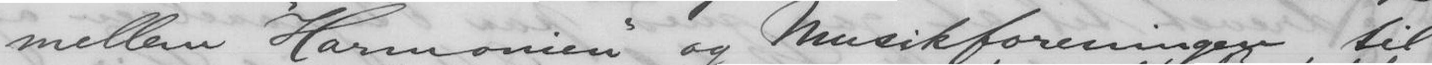mellem "Harmonien" og Musikforeningen til
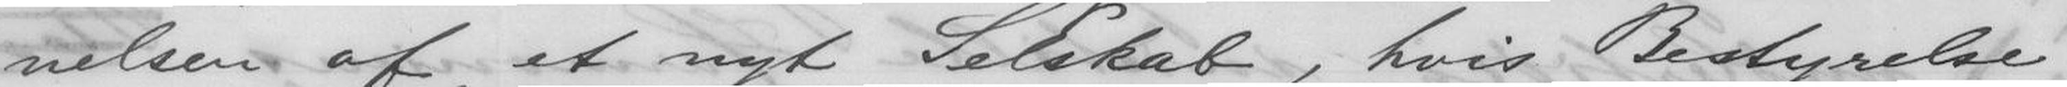nelsen af et nyt Selskab, hvis Bestyrelse
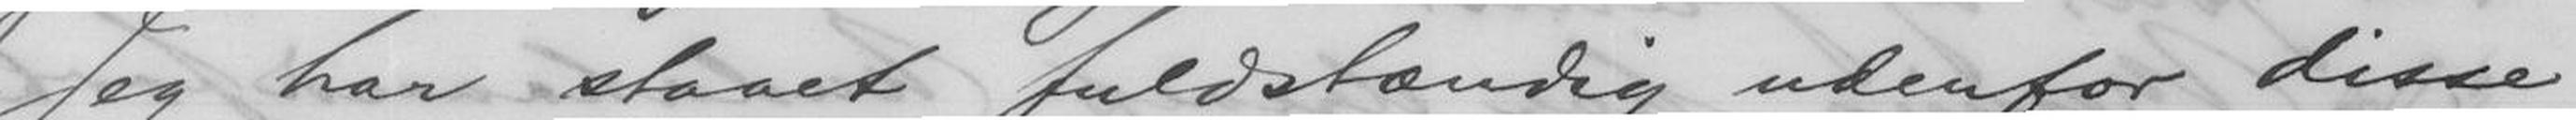Jeg har staaet fuldstændig udenfor disse
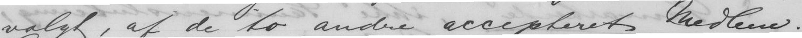valgt, af de to andre accepteret Medlem.
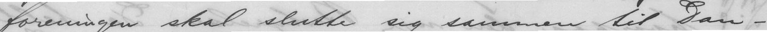foreningen skal slutte sig sammen til Dan-
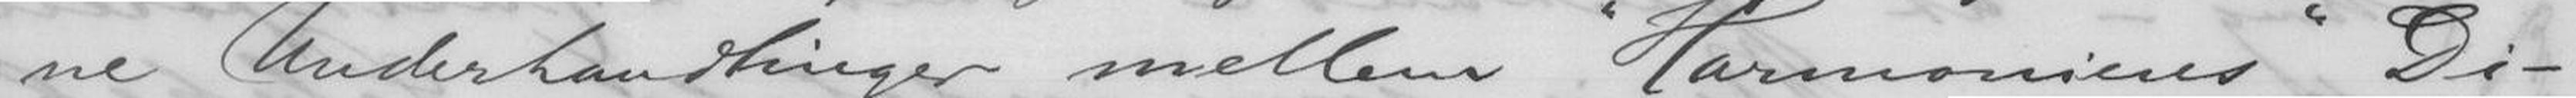ne Underhandlinger mellem "Harmoniens" Di-
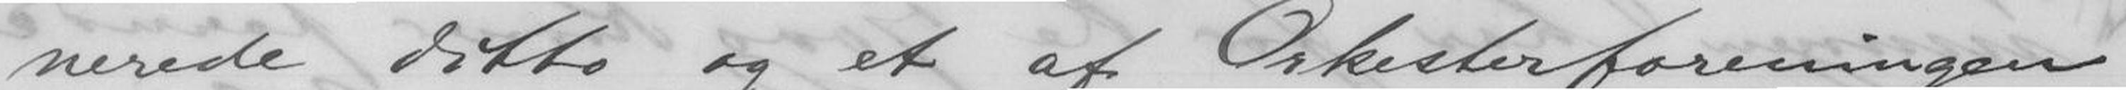nerede ditto og et af Orkesterforeningen
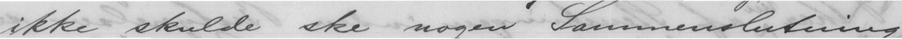ikke skulde ske nogen Sammenslutning
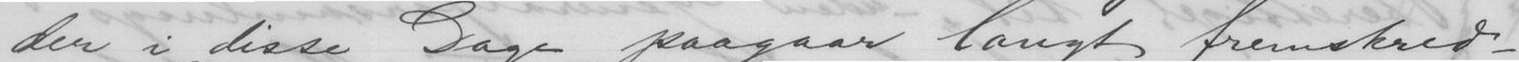der i disse Dage paagaar langt fremskred-
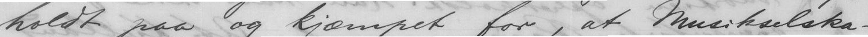holdt paa og kjæmpet for, at Musikselska-
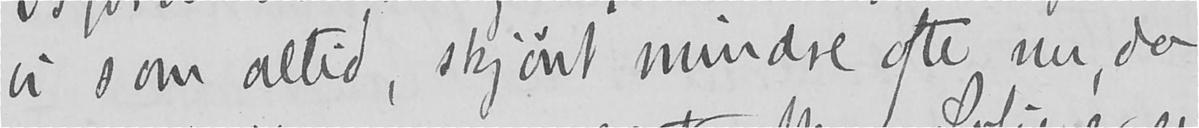vi som altid, skjønt mindre ofte nu, da
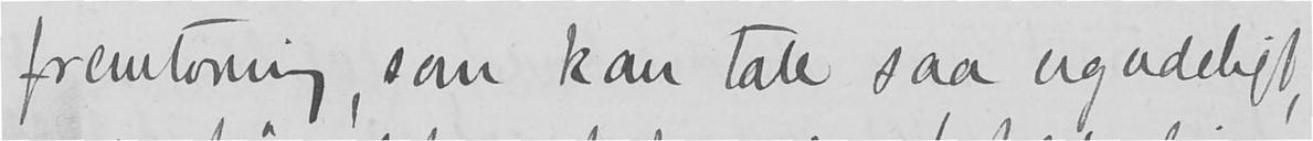fremtoning, som kan tale saa ugudeligt,
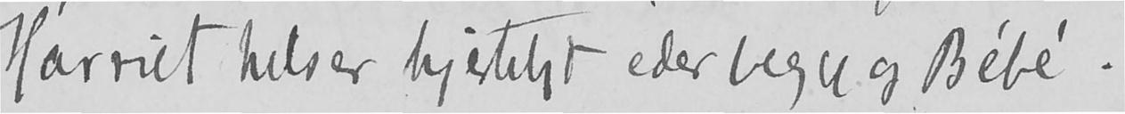Harriet hilser hjerteligt eder begge og Bébé.
